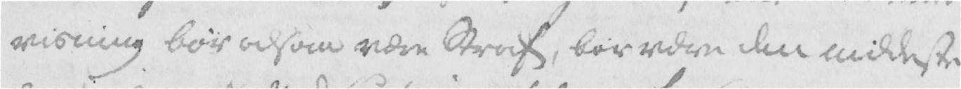visning bør altsaa være Straf, bør være den mildeste
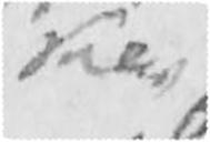selv
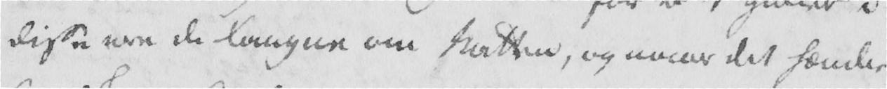disse ere de Fangne om Natten, og naar det hænder
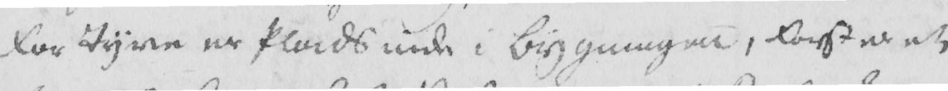For Tyve er Plads inde i Bygningen, først er et
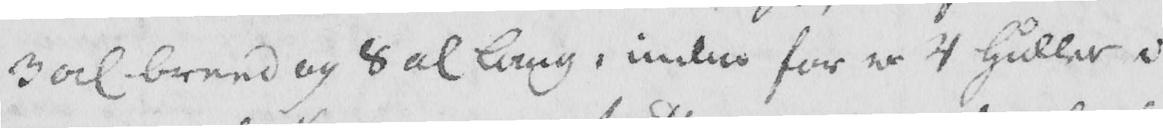3 al breed og 8 al lang, inden for er 4 Huller i
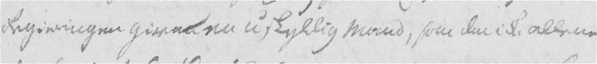Regieringen give en uskyldig Mand, som den icke allene
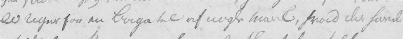20 Uger for en Bagatel af nogle Mark, hvad der havde
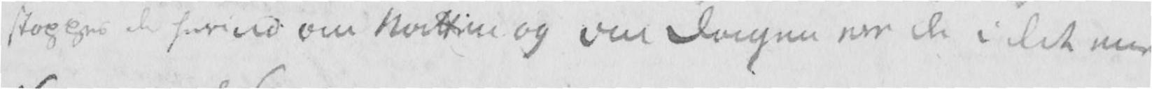stoppes de herind om Natten og om Dagen ere de i det ene
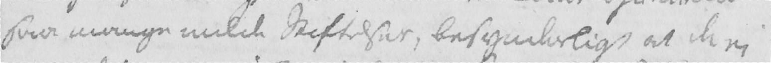saa mange milde Stiftelser, besynderligt at der ei
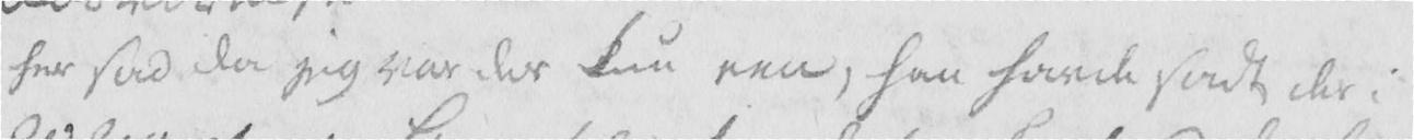Her sad da jeg var der kun een, han havde sadt der
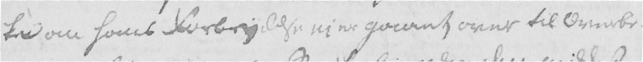ke om hans Forbrydelse ej er gaaet over til Overbe-
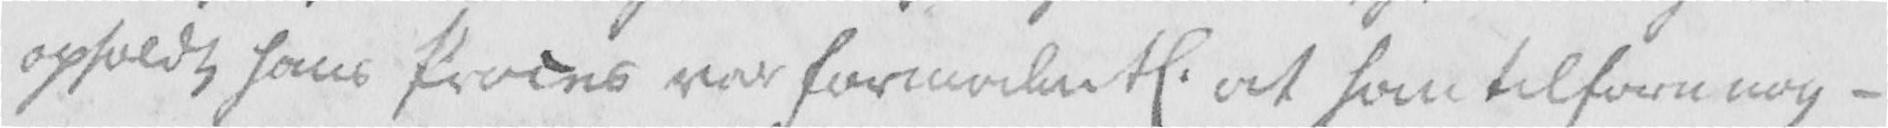opholdt hans Proces var formodentl. at han tilforn nog-
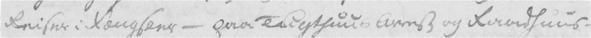Reiser i Fængsler - paa Tugthuus Arrest og Raadhuus-
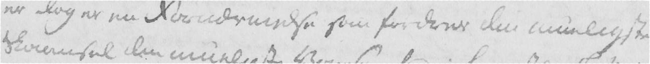er dog er en Fornærmelse som fordrer den mueligste
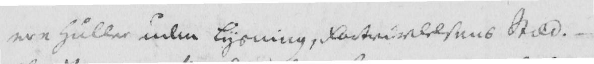ere Huller uden Lysning, Fortvivlelsens Stæd. -
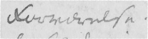Forværelse.
